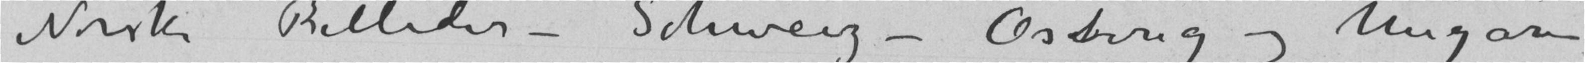af Norske Billeder – Schweitz – Østerig og Ungarn
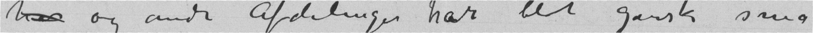har og andre Afdelinger har blot ganske små
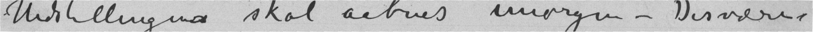Udstillingen skal aabnes imorgen – Desværre
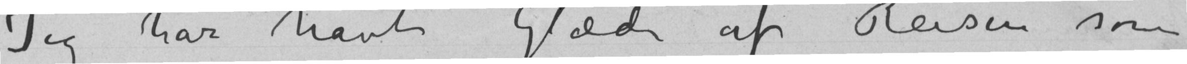Jeg har havt Glæde af Reisen som
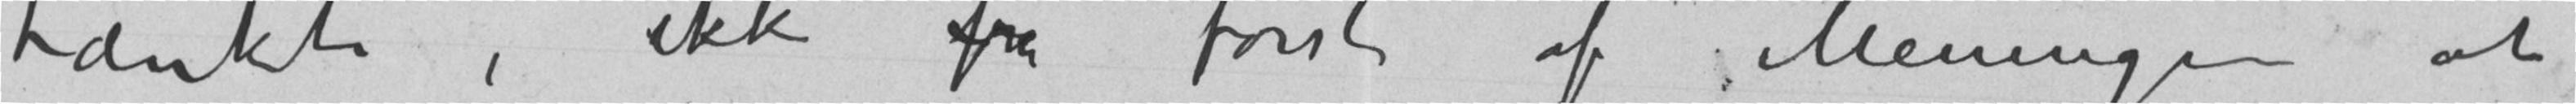tænkte, ikke fra først af Meningen at
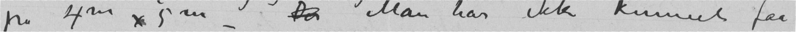på 4m x 5m – Der Man har ikke kunnet faa
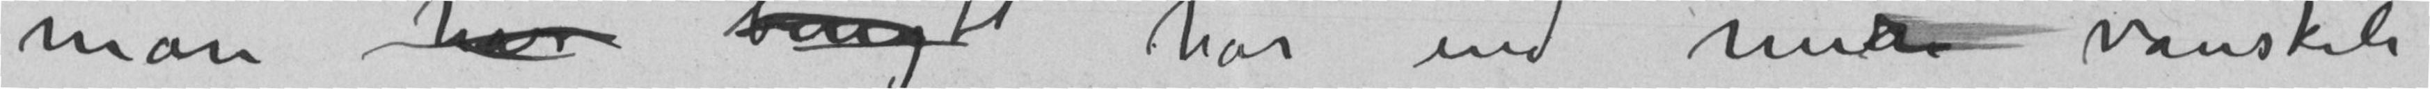man har brug til har end mer vanskeli
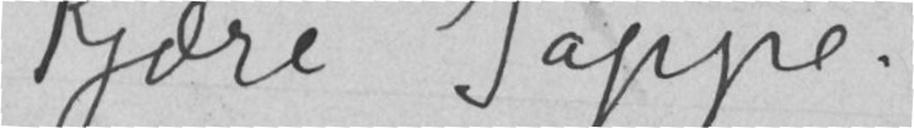Kjære Jappe.
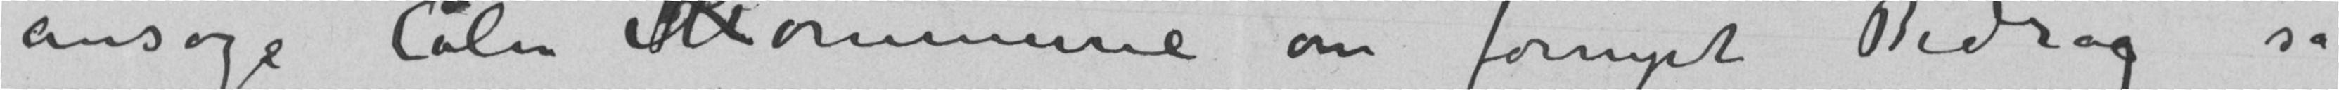ansøge Cöln Kommune om fornyet Bidrag så
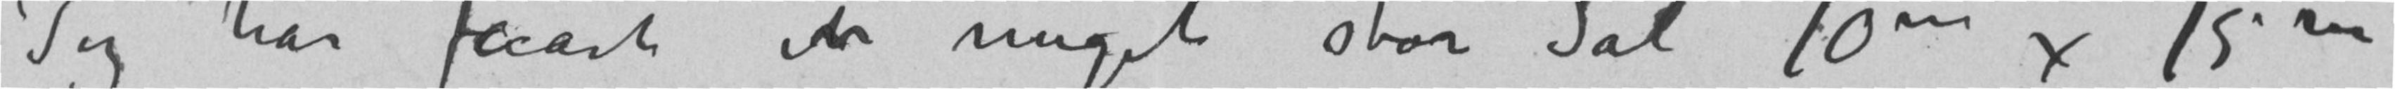Jeg har faaet en meget stor Sal 10m x 15m
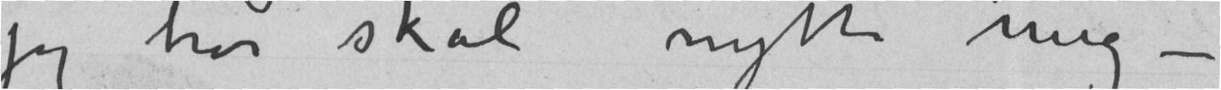jeg tror skal nytte mig –
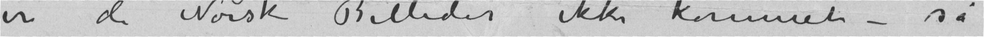er de Norske Billeder ikke kommet – så
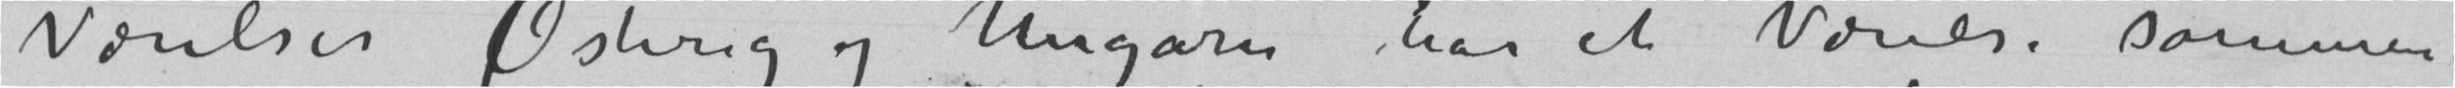VærelserØsterig og Ungarn har et Værelse sammen
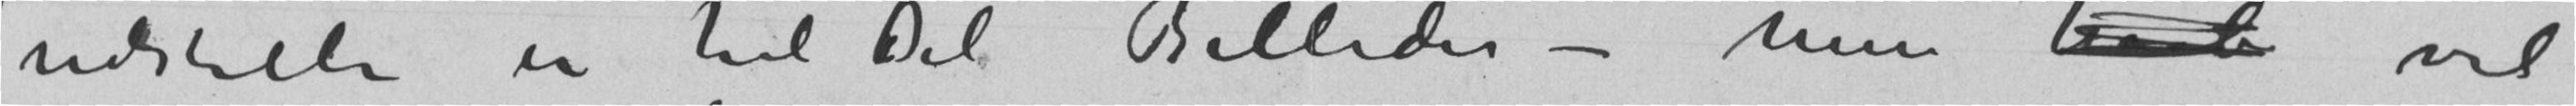udstille en hel Del Billeder – men haab vil
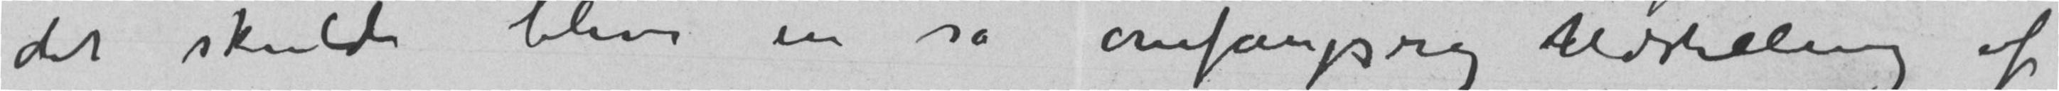det skulde blive en så omfangsrig Udstilling
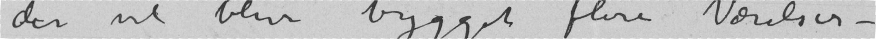der vil blive bygget flere Værelser –
